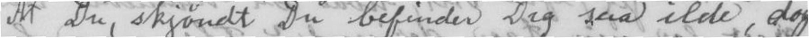At Du, skjøndt Du befinder Dig saa ilde dog
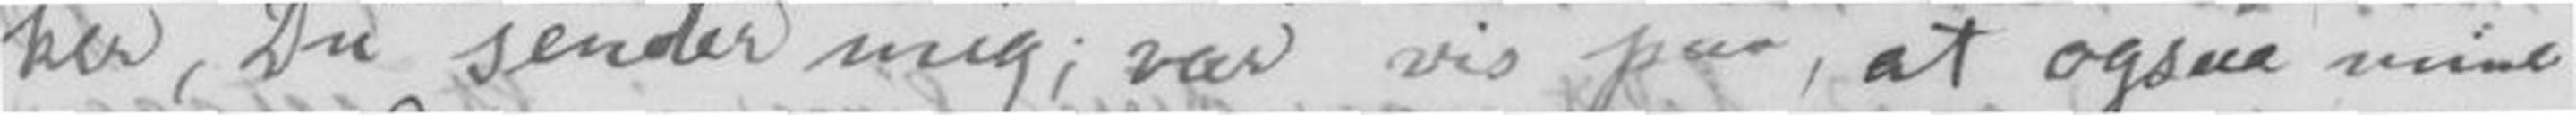ker, Du sender mig, vær vis paa, at ogsaa mine
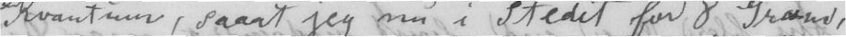Kvantum, saa at jeg nu i Stedet for 8 Gram,
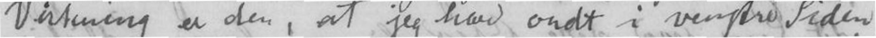Virkning er den, at jeg har indt i venstre Siden;
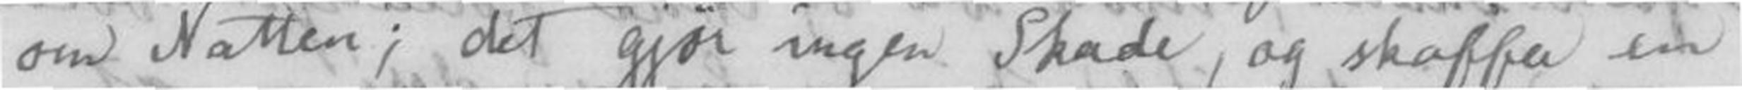om Natten; det gjør ingen Skade, og skaffer en
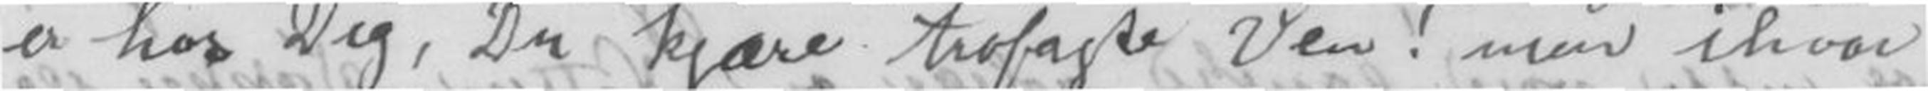er hos Dig, Du kjære trofaste Ven! men ihvor
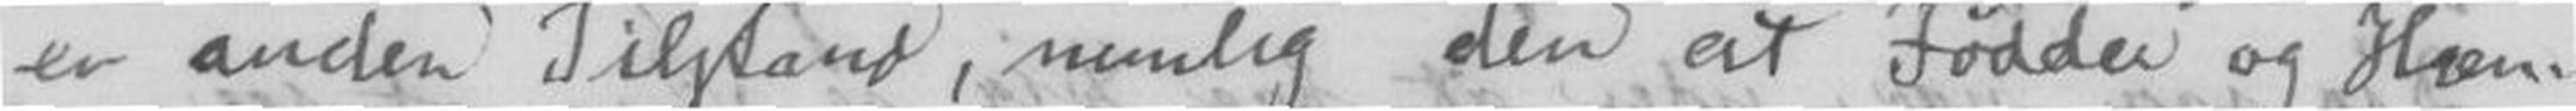en anden Tilstand, nemlig den at Fødder og Hæn-
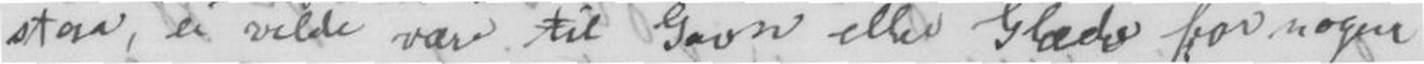staar, ei vilde være til Gavn eller Glæde for nogen
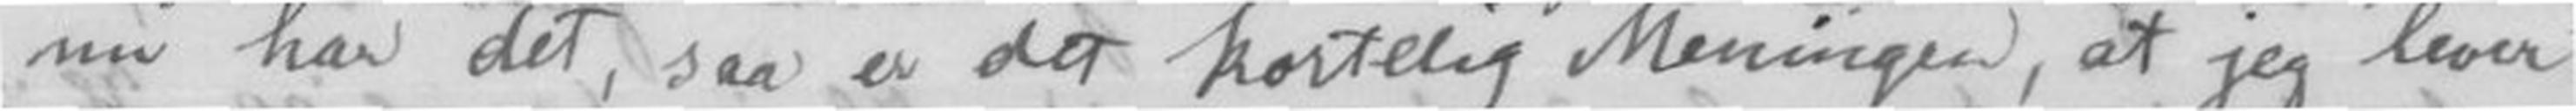nu har det, saa er det kortelig Meningen, at jeg levet
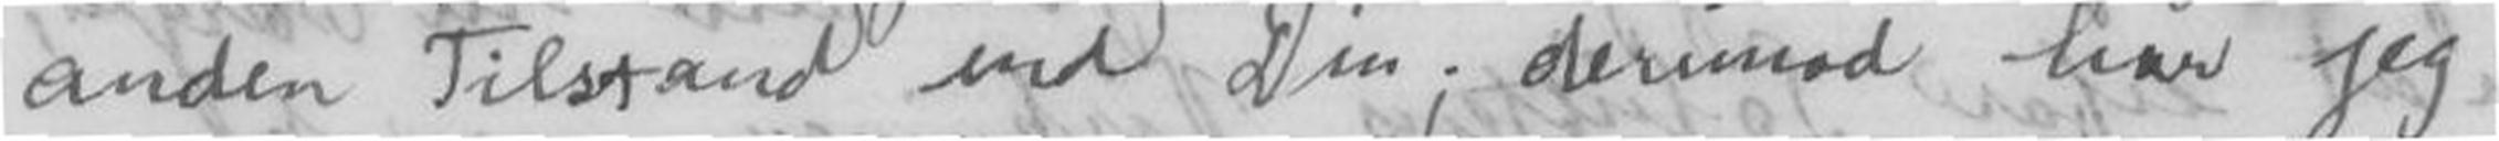anden Tilstand end Din; derimod har jeg
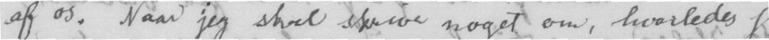af os. Naar jeg skal skrive noget om, hvorledes jeg
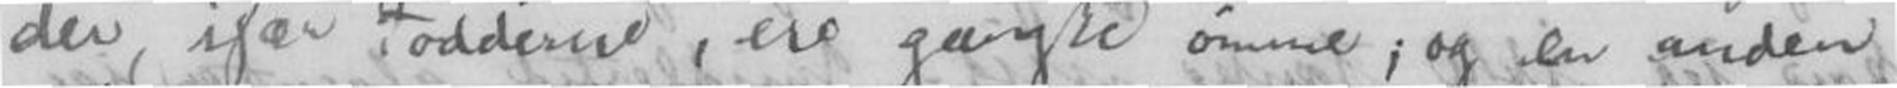der, især Fødderne, ere ganske ømme; og en anden
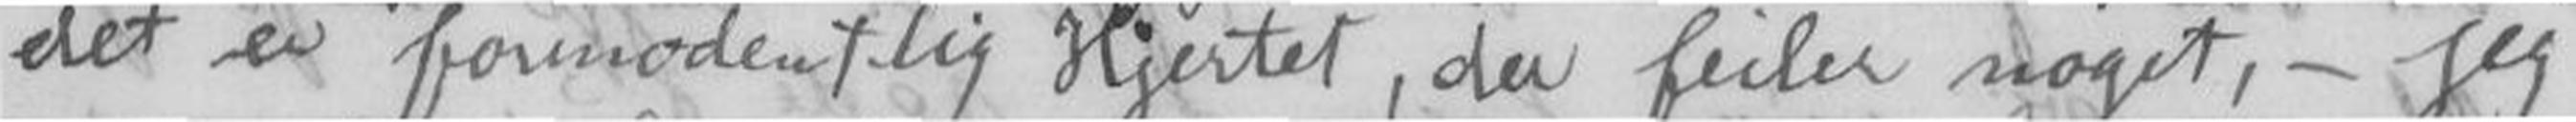det er formodentlig Hjertet, der feiler noget, - jeg
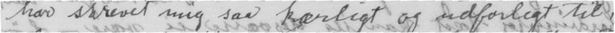har skrevet mig saa kærligt og udførligt til,
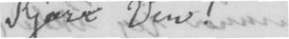Kjære Ven!
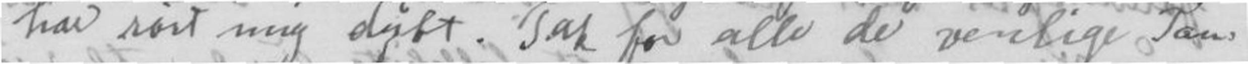har rørt mig dybt. Tak for alle de venlige Tan-
